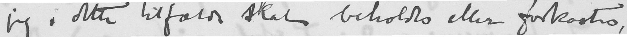jeg i dette tilfælde skal beholdes eller forkastes,
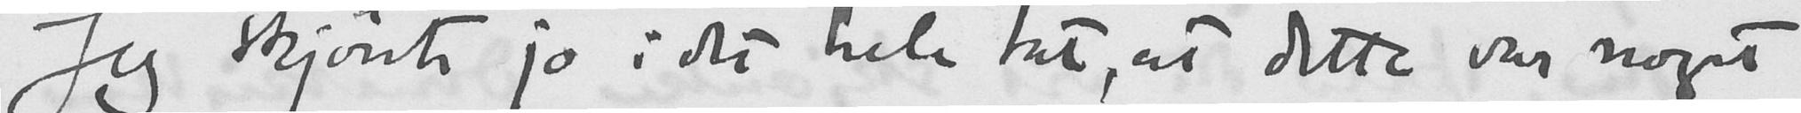Jeg skjønte jo i det hele tat, at dette var noget
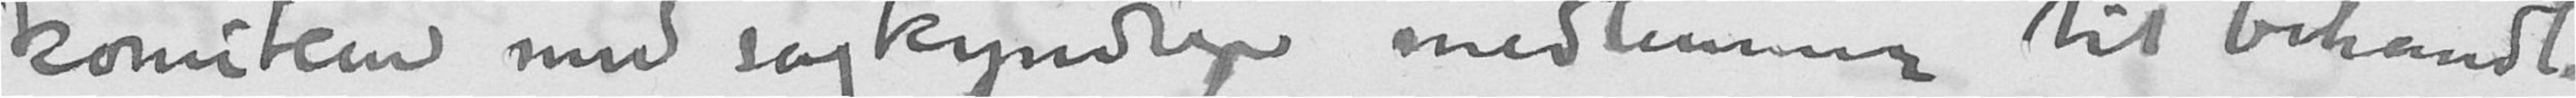komiteen med sagkyndige medlemmer til behandl.
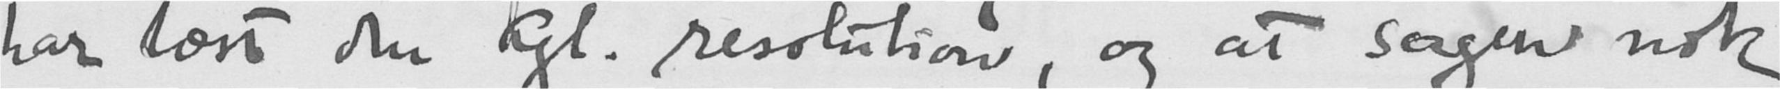har læst den Kgl. resolution, og at sagen nok
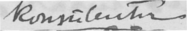konsulenter
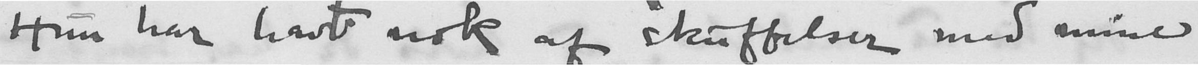Hun har havt nok af skuffelser med mine
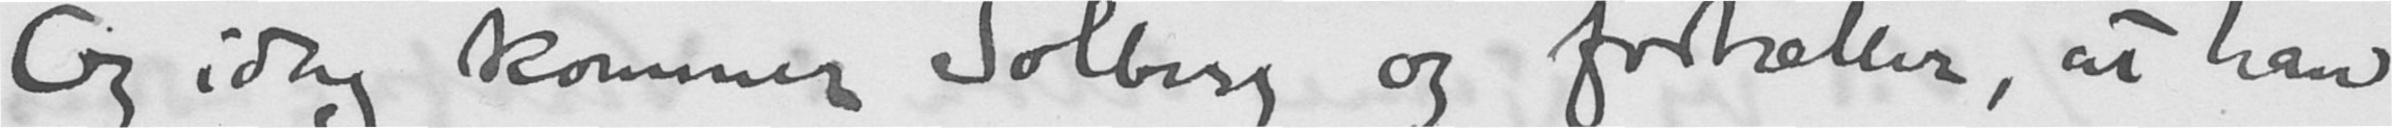Og idag kommer Solberg og fortæller, at han
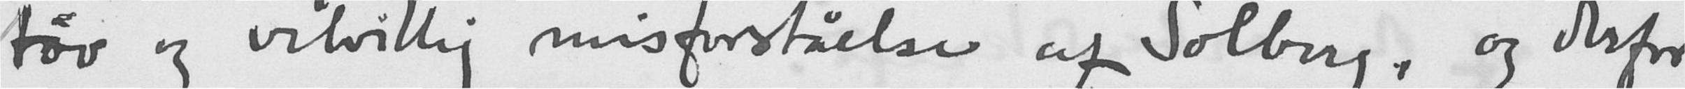tøv og velvillig misforståelse af Solberg, og derfor
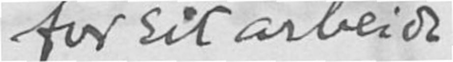for sit arbeide
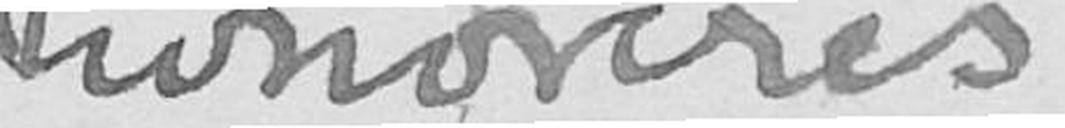honoreres
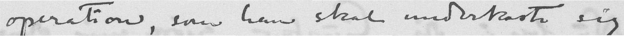operation, som han skal underkaste sig
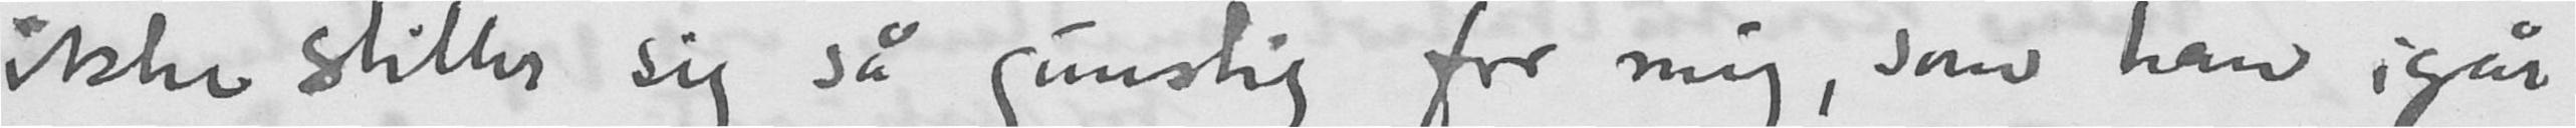ikke stiller sig så gunstig for mig, som han igår
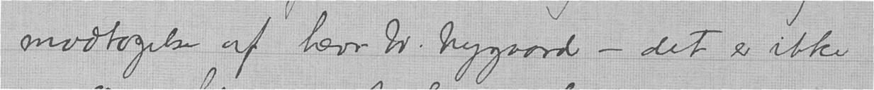modtagelse af herr W. Nygaard — det er ikke
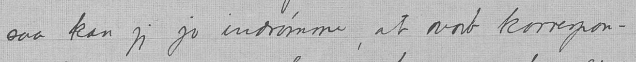saa kan jeg jo indrømme, at vort korrespon-
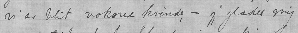vi er blit voksne kvinder, — jeg glæder mig
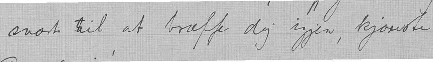snart til at træffe dig igjen, kjæreste
